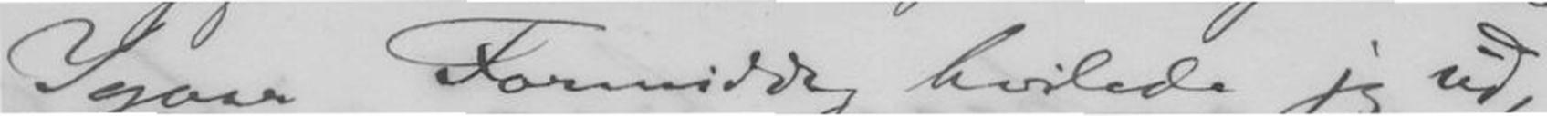Igaar Formiddag hvilede jeg ud,
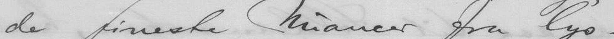de fineste Nuancer fra lys-
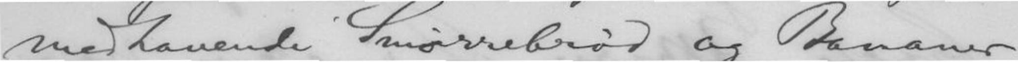medhavende Smørrebrød og Bananer
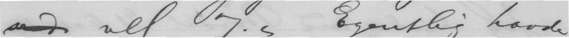ved vel 7. Egentlig havde
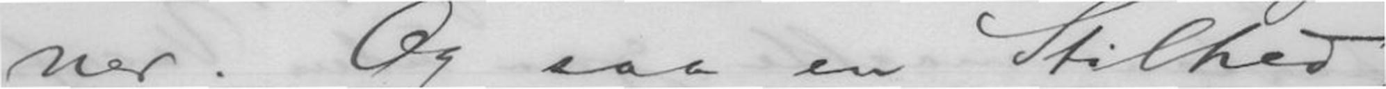ner. Og saa en Stilhed,
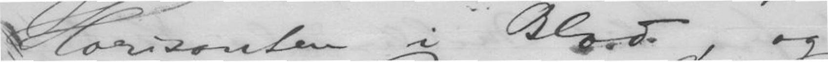Horisonten i Blod, og
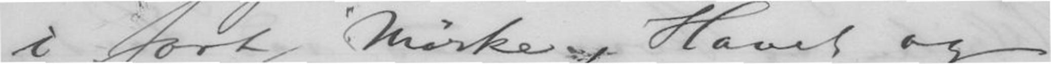i sort Mørke, Havet og
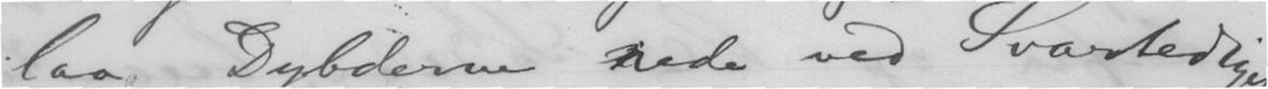laa Dybderne nede ved Svartediget
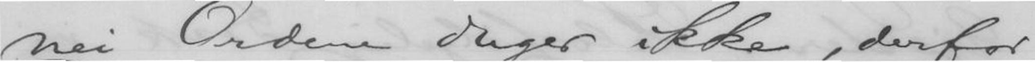nei Ordene duger ikke, derfor
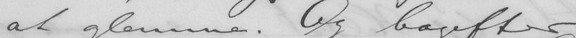at glemme. Og bagefter
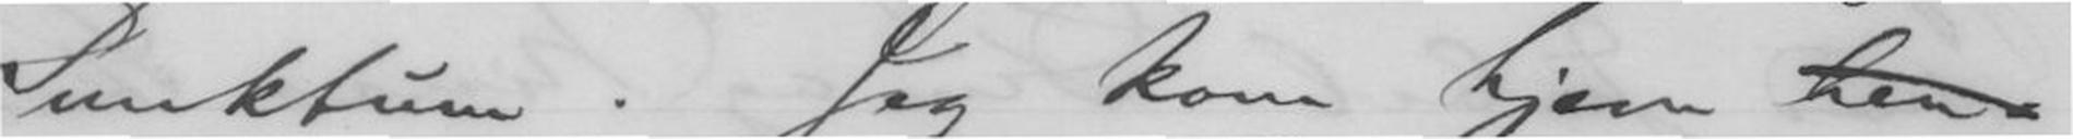Punktum. Jeg kom hjem hen-
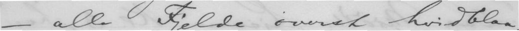- alle Fjelde øverst hvidblaa,
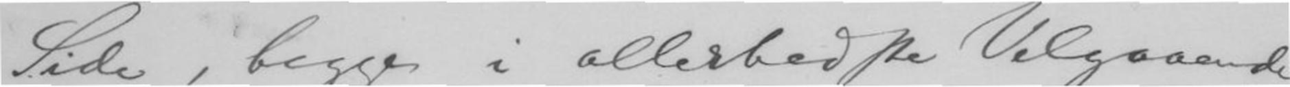Side, begge i allerbedste Velgaaende.
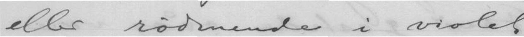eller rødmende i violet
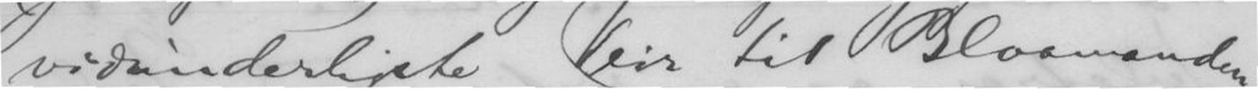vidunderligste Veir til Blaamanden
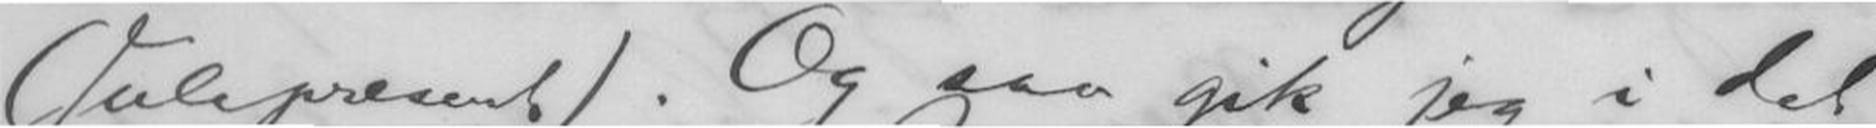(Julepresent). Og saa gik jeg i det
